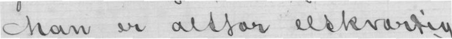Man er altfor elskværdig
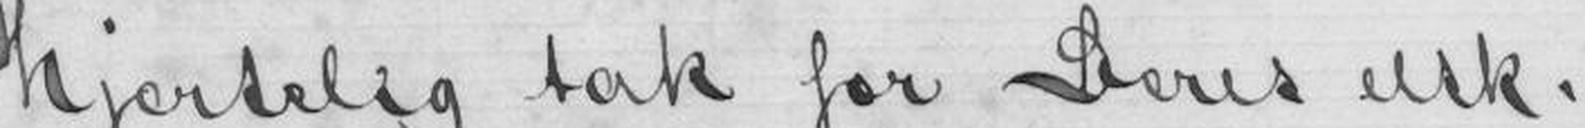Hjertelig tak for Deres elsk-
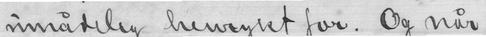umådelig henrykt for. Og når
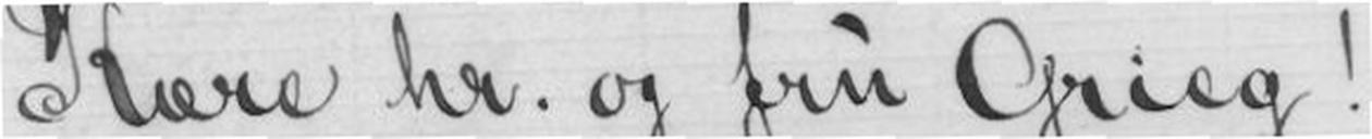Kære hr. og fru Grieg!
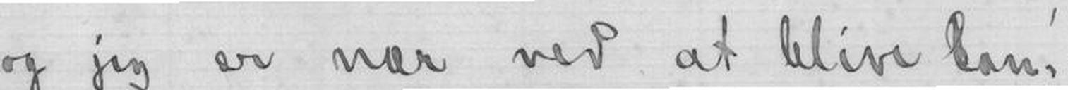og jeg er nær ved at blive ban-
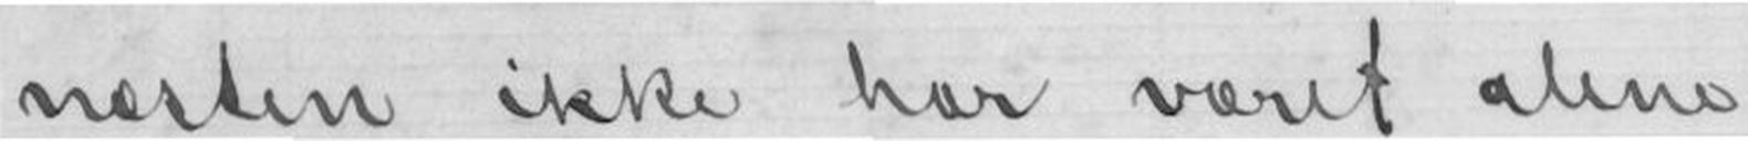næsten ikke har været alene
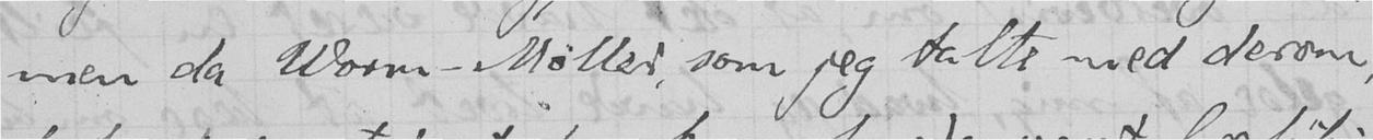men da Worm-Møller, som jeg talte med derom,
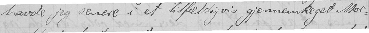havde jeg senere i et tilfældigvis gjennemkeget Mor-
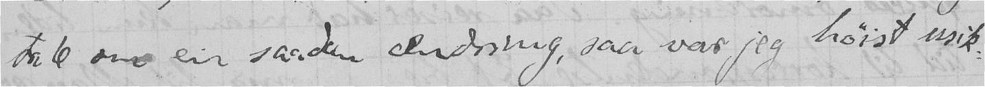tale om en saadan ændring, saa var jeg høist usik-
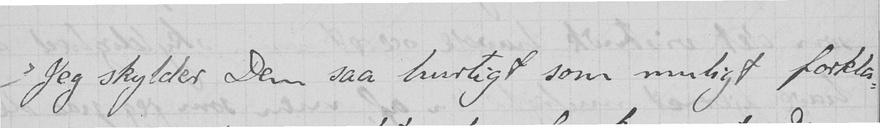Jeg skylder Dem saa hurtigt som muligt forkla-
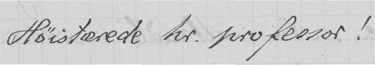Høistærede hr. professor!
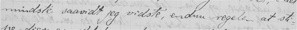mindste saavidt jeg vidste, endnu regelen at sti-
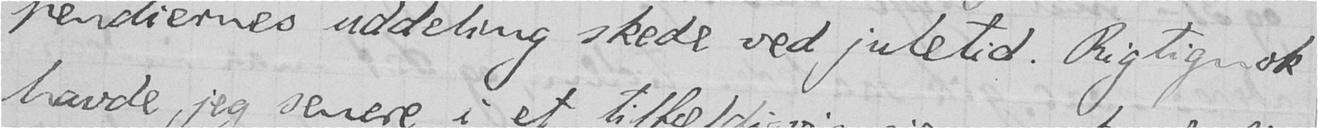pendiernes uddeling skede ved juletid. Rigtignok
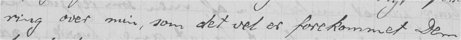ring over min, som det vel er forekommet Dem
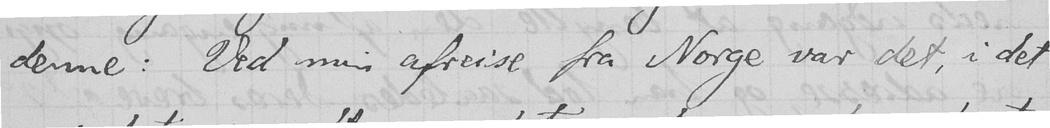denne: Ved min afreise fra Norge var det, i det
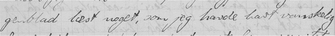genblad læst noget, som jeg havde havt vanskelig
